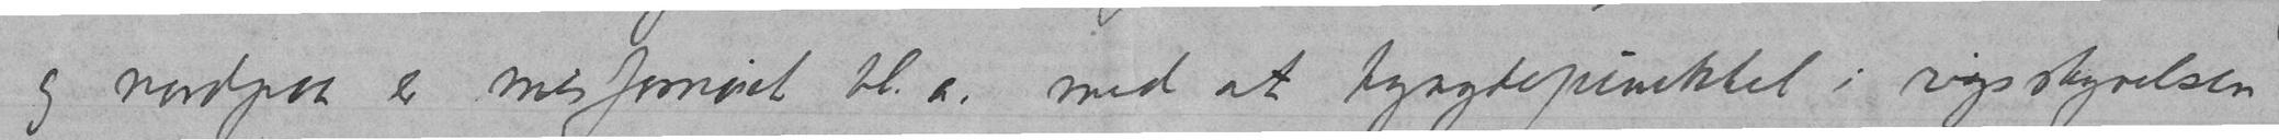og nordpaa er misfornøiet bl.a. med at tyngdepunktet i rigsstyrelsen
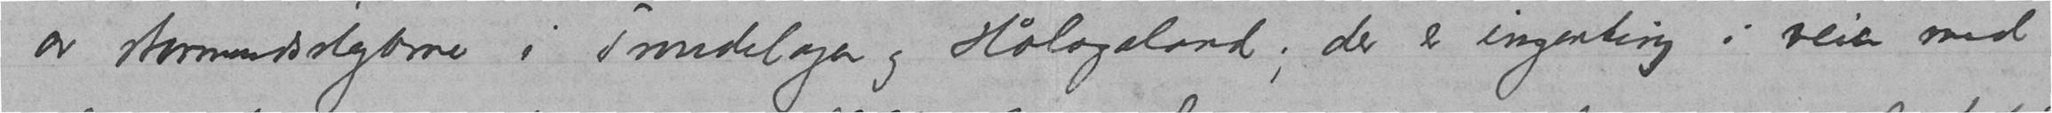av stormandsslegterne i Trøndelagen og Hålogaland; der er ingenting i veien med
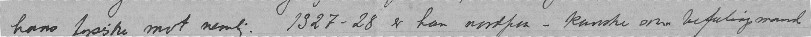hans fysiske mot nemlig. 1327-28 er han nordpaa – kanske som befalingsmand
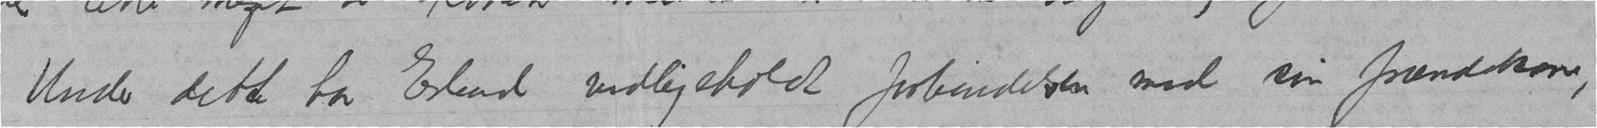Under dette har Erlend vedligeholdt forbindelsen med sin frændekone,
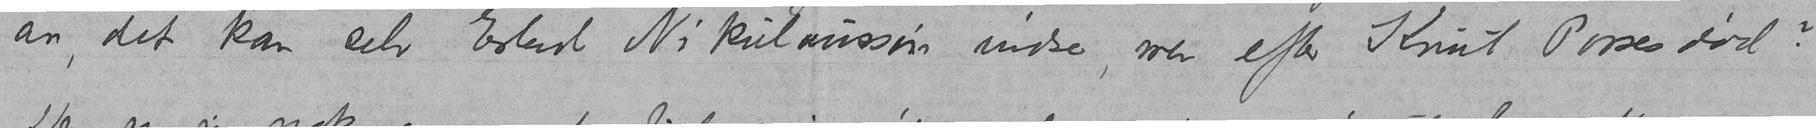an, det kan selv Erlend Nikulaussøn indse, men efter Knut Porses død?
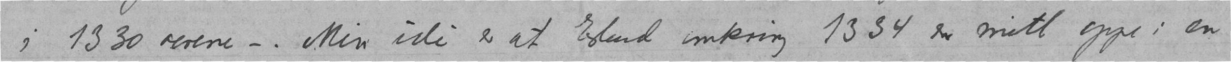i 1330 aarene –. Min idé er at Erlend omkring 1334 er mitt oppe i en
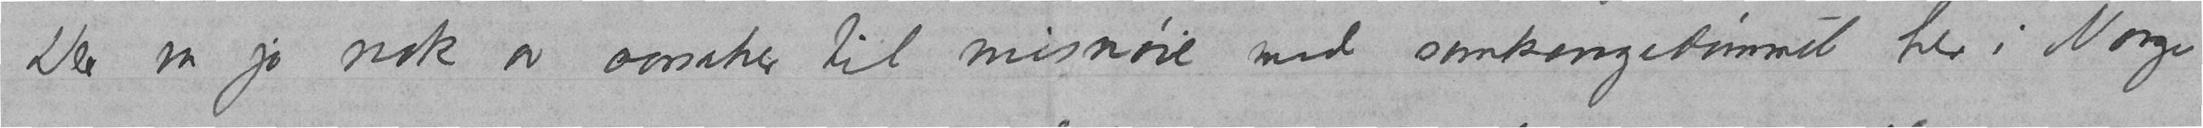Der var jo nok av aarsaker til misnøie med samkongedømmet her i Norge
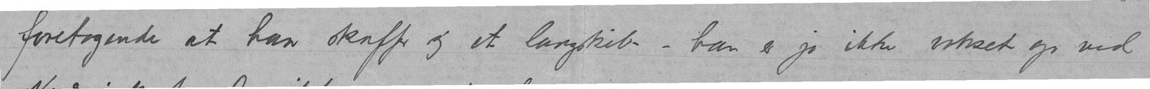foretagende at han skaffer sig et langskib – han er jo ikke vokset op ved
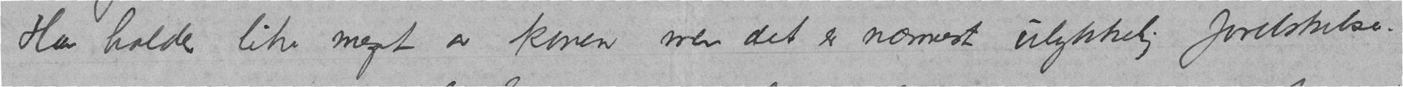Han holder like meget av konen men det er nærmest ulykkelig forelskelse.
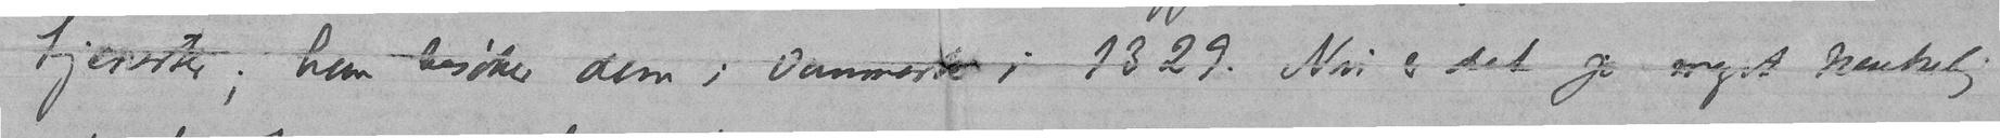tjenester; han besøker dem i Danmark i 1329. Nu er det jo meget tænkelig
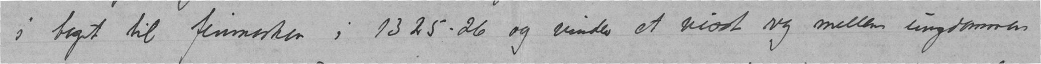i toget til finmarken i 1325-26 og vinder et visst ry mellem ungdommen
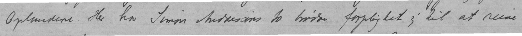Oplandene. Her har Simon Andressøns to brødre forpligtet sig til at reise
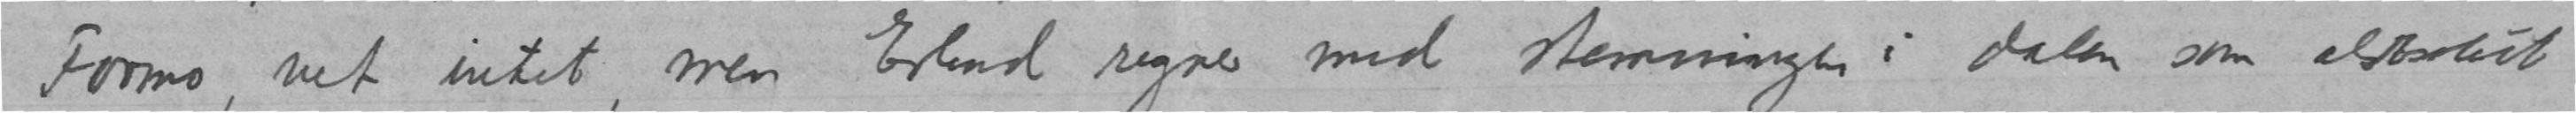Formo, vet intet, men Erlend regner med stemningen i dalen som absolut
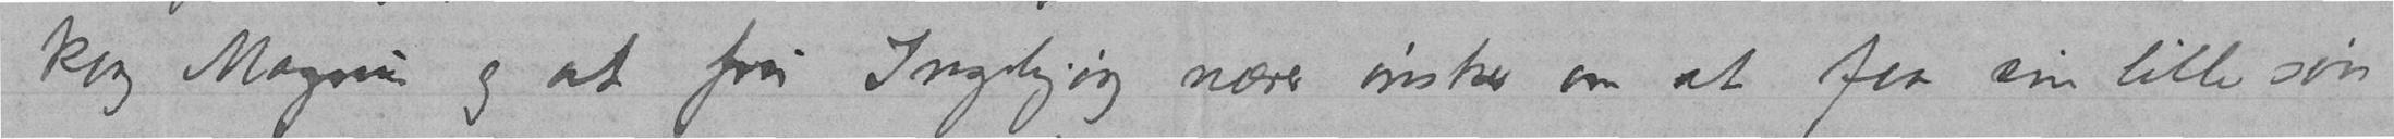kong Magnus og at fru Ingebjørg nærer ønske om at faa sin lille søn
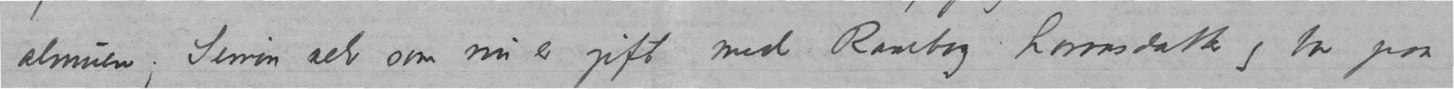almuen; Simon selv som nu er gift med Ramborg Lavransdatter og bor paa
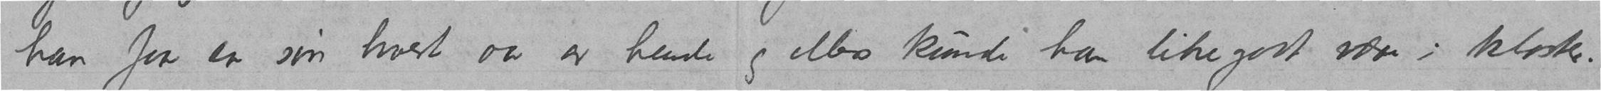han faar en søn hvert aar av hende og ellers kunde han like godt være i kloster.
